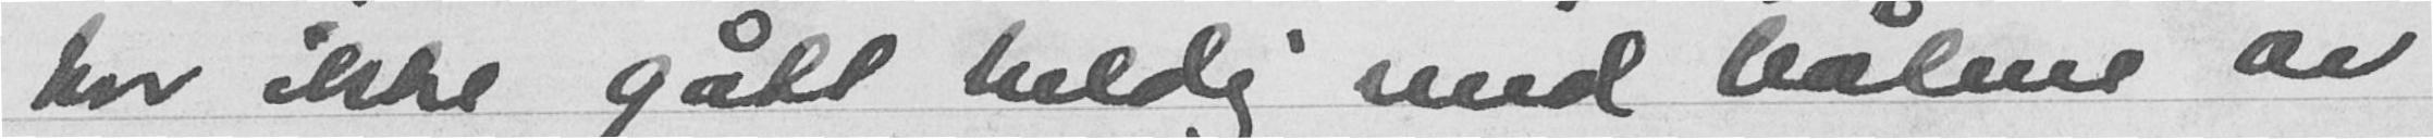har ikke gått heldig med båten av
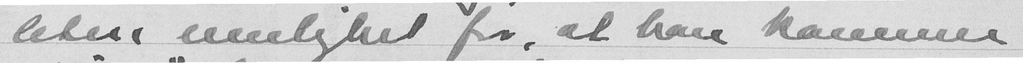liten mulighet for, at han kommer
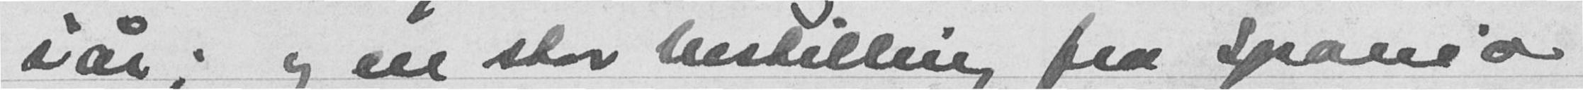iår; og en stor bestilling fra Spania
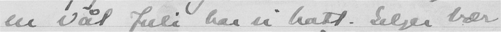en våt Juli har vi hatt. Selger bær
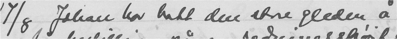17/8 Johan har hatt den store gleden å
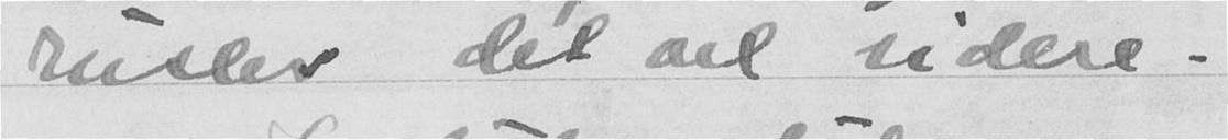rusler det vel videre.
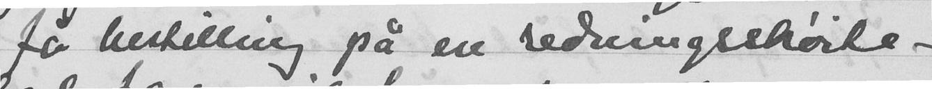få bestilling på en redningeskøite -
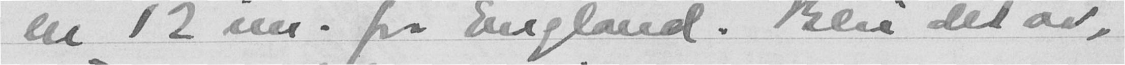en 12 m. fra England. Blir det av,
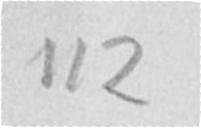112
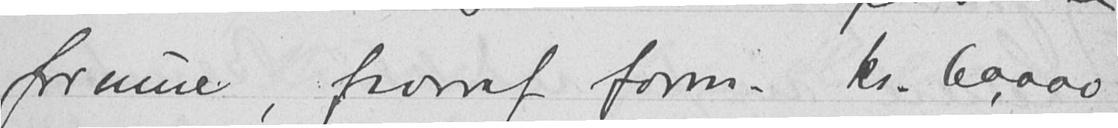formue, hvoraf form. kr. 60,000
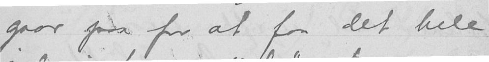gaar paa for at faa det hele
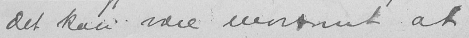det kan være morsomt at
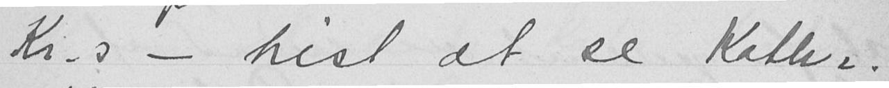Kr.s - trist at se Katti,
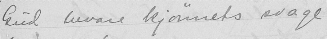Gud bevare kjønnets svage
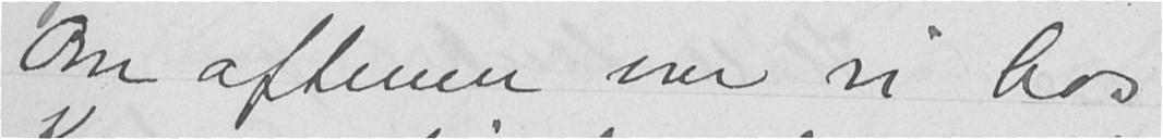Om aftenen var vi hos
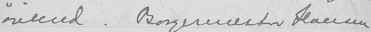øiemed. Borgermester Hansen
